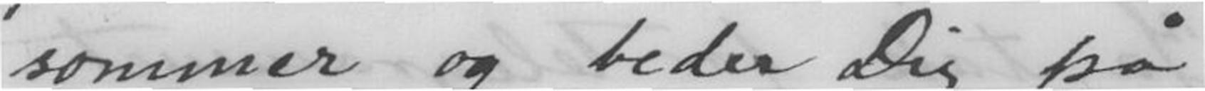sommer og beder Dig på
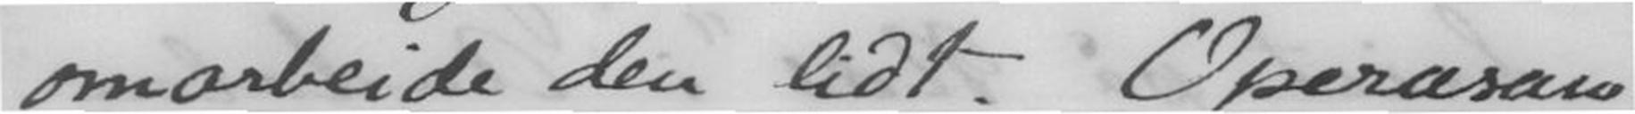omarbeide den lidt. Operasan-
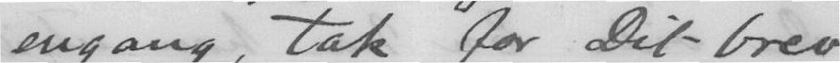engang, tak for Dit brev
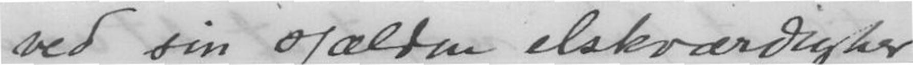ved sin sjældne elskværdighed.
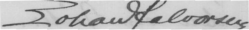Johan Halvorsen
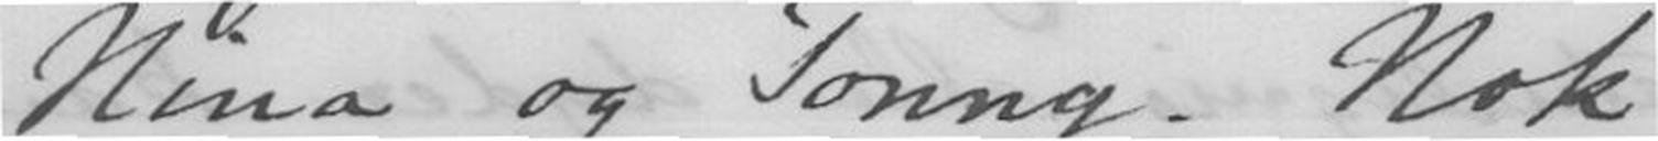Nina og Tonny. Nok
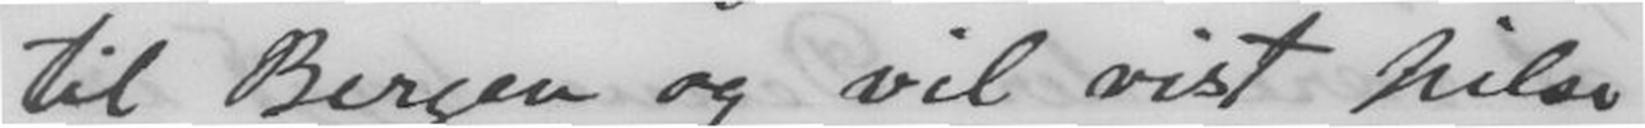til Bergen og vil vist hilse
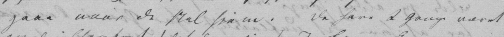gaae naar de skal hjem. De have 2 Gange været
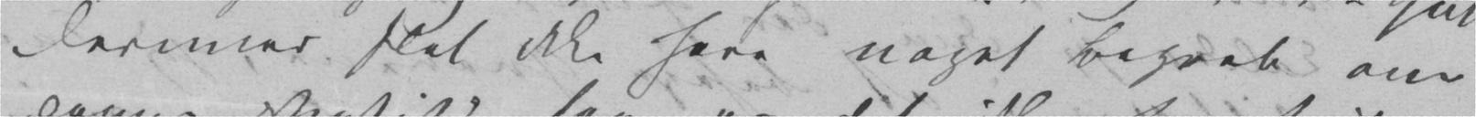derimod slet ikke have noget Begreb om
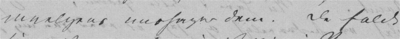mueligens mishager Dem. De faldt
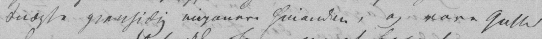Knægte gjensidig imponere hinanden, og vore Gutter
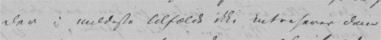der i mildeste Tilfælde ikke intreserer Dem,
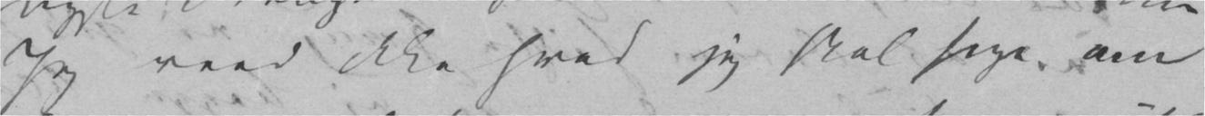Jeg veed ikke hvad jeg skal sige om
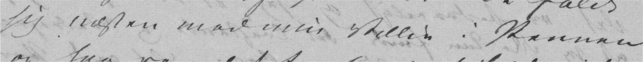sig næsten mod min Villie i Pennen
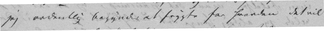jeg ordentlig begynder at frygte for hvordan det vil
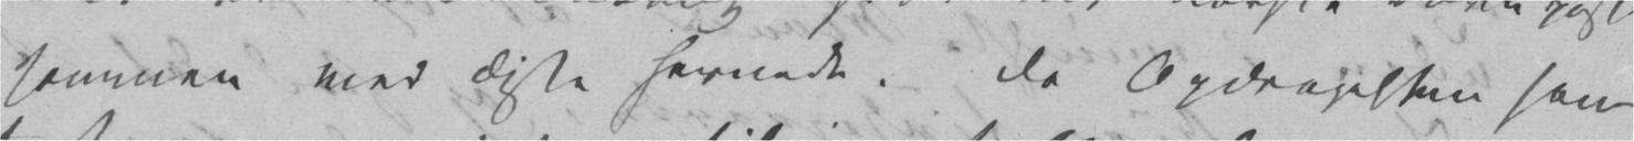sammen med disse hernede. Da Opdragelsen som
